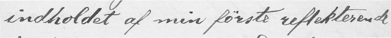indholdet af min første reflekterende
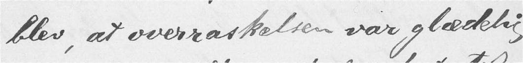blev, at overraskelsen var glædelig,
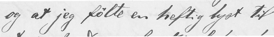og at jeg følte en heftig lyst til
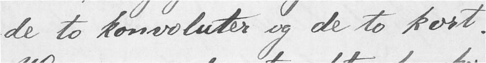de to konvoluter og de to kort.
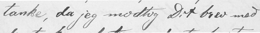tanke, da jeg modtog Dit brev med
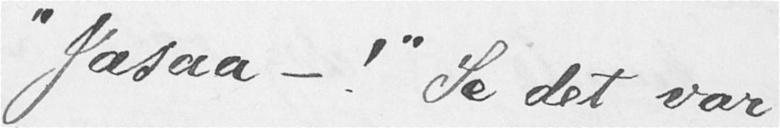"Jasaa –!" Se det var
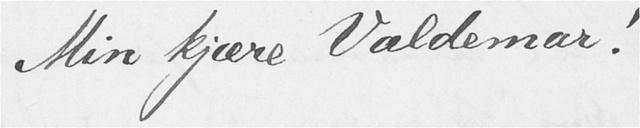Min kjære Valdemar!
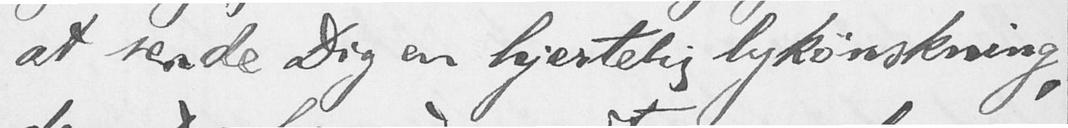at sende Dig en hjertelig lykønskning,
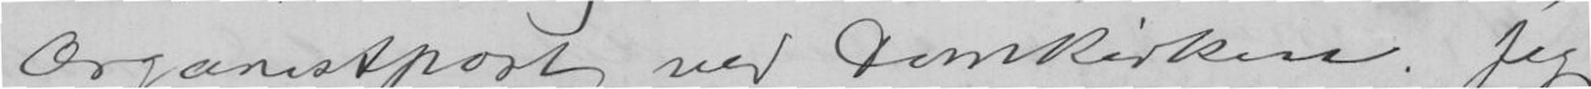Organistpost ved Domkirken. Jeg
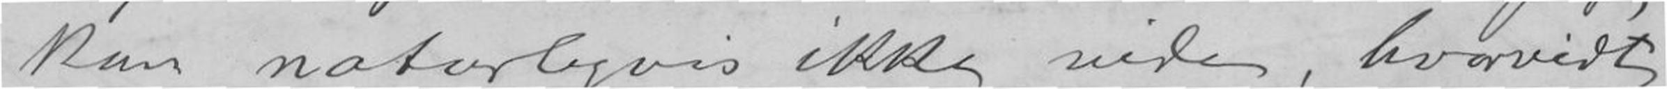kan naturligvis ikke vide, hvorvidt
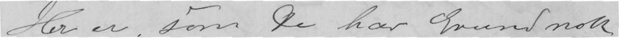Her er, som De har Grund nok
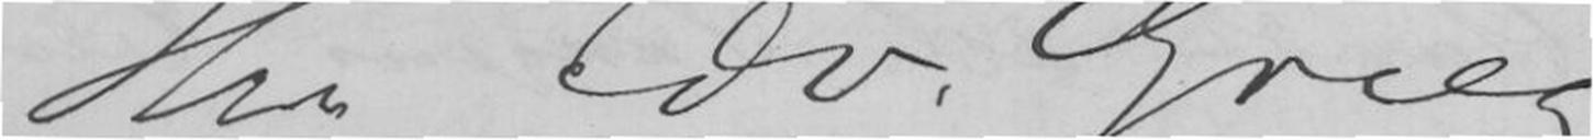Herr Edv. Grieg.
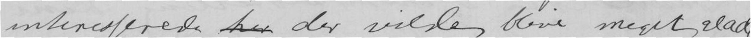interesserede, der vilde blive meget glade,
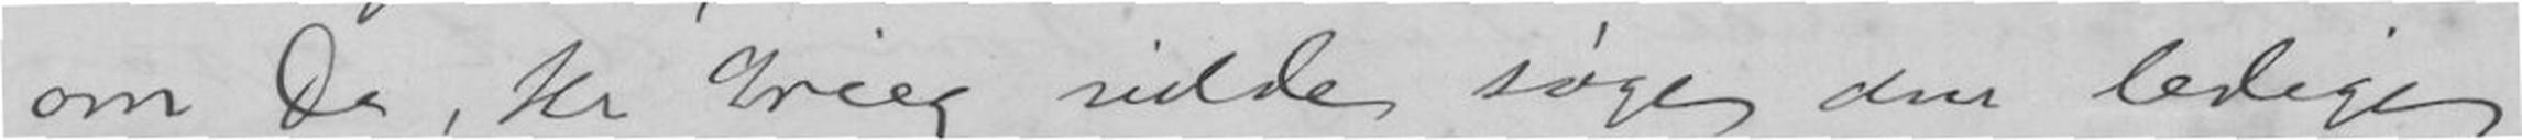om De, Hr Grieg vilde søge den ledige
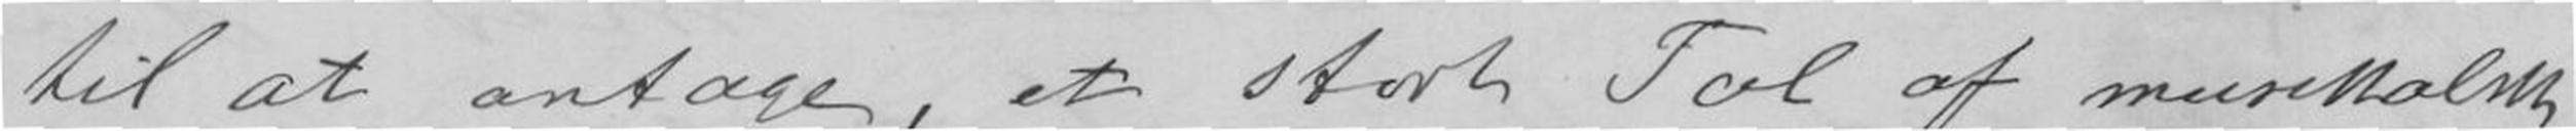til at antage, et stort Tal af musikalske
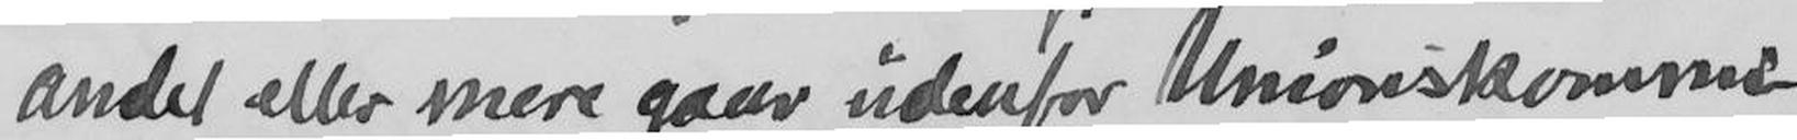andet eller mere gaar udenfor Unionskommi-
